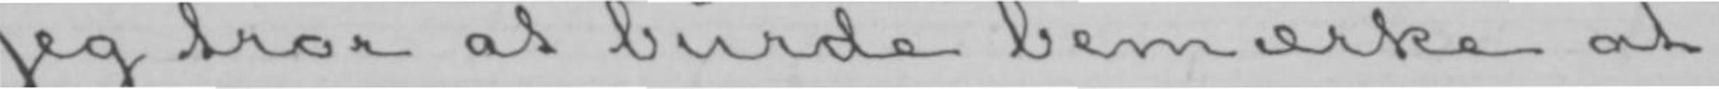Jeg tror at burde bemærke at
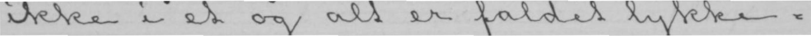ikke i et og alt er faldet lykke-
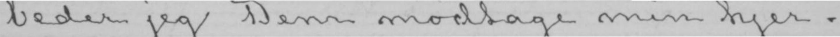beder jeg Dem modtage min hjer-
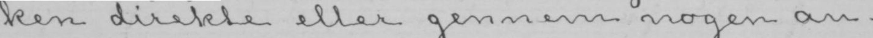ken direkte eller gennem nogen an-
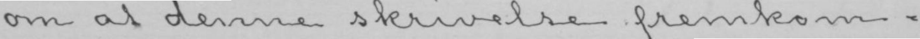om at denne skrivelse fremkom-
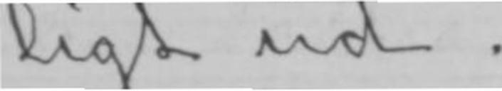ligt ud.
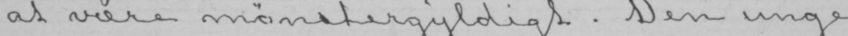at være mønstergyldigt. Den unge
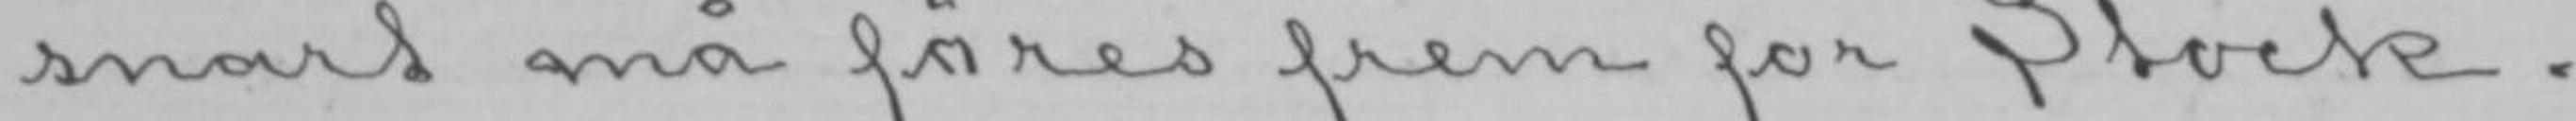snart må føres frem for Stock-
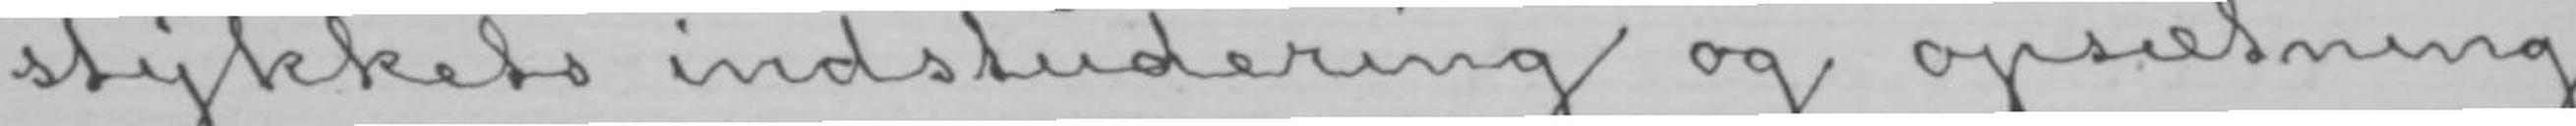stykkets indstudering og opsætning
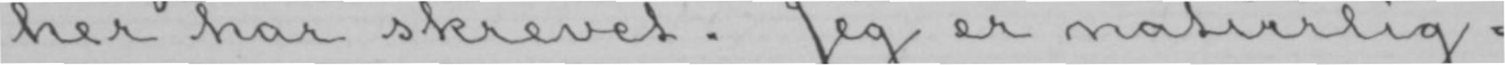her har skrevet. Jeg er naturlig-
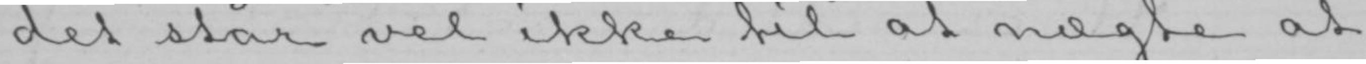det står vel ikke til at nægte at
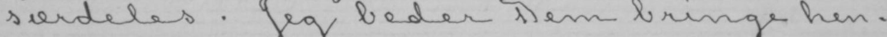særdeles. Jeg beder Dem bringe hen-
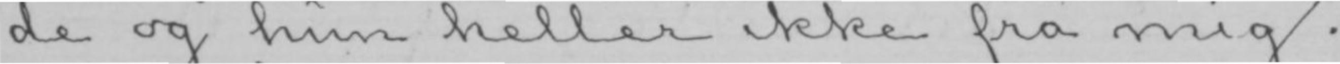de og hun heller ikke fra mig.
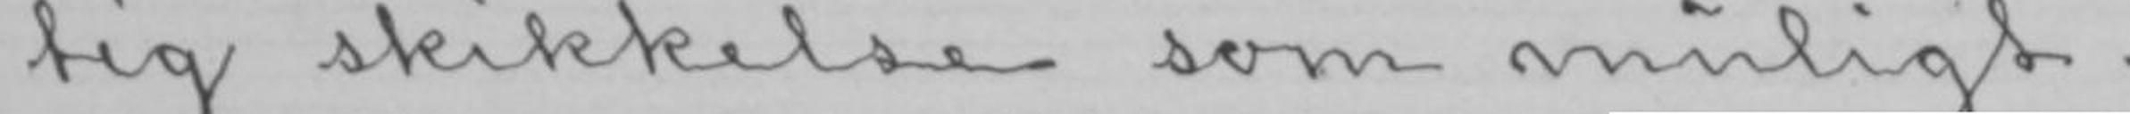tig skikkelse som muligt.
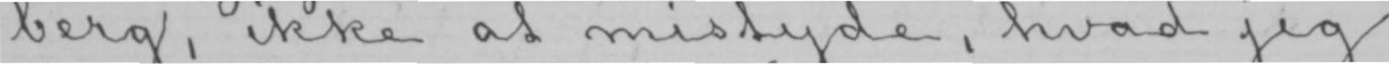berg, ikke at mistyde, hvad jeg
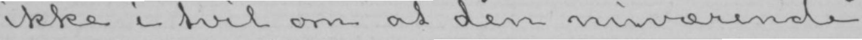ikke i tvil om at den nuværende
